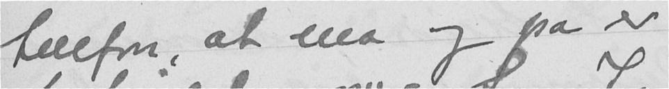telefon, at ma og pa er
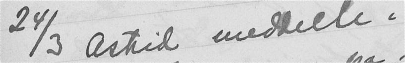24/3 Astrid meddelte i
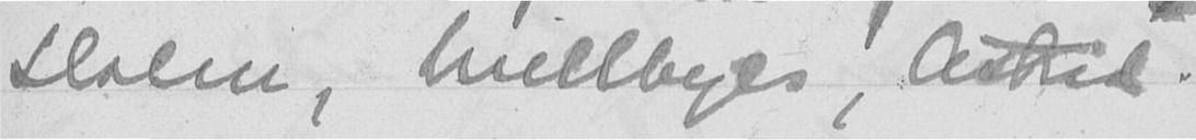Holm, Mellbyes, Astrid.
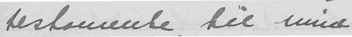testamente til mine
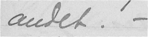andet. -
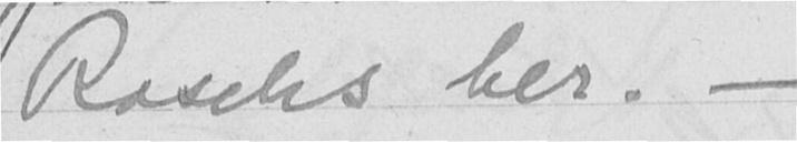Raschs her. -
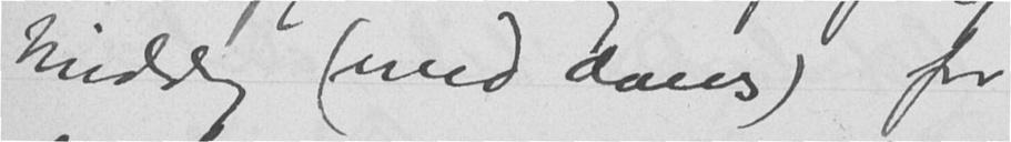Middag (med dans) for
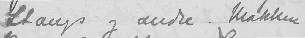Stangs og andre. Makken
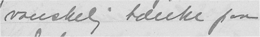vanskelig tænke paa
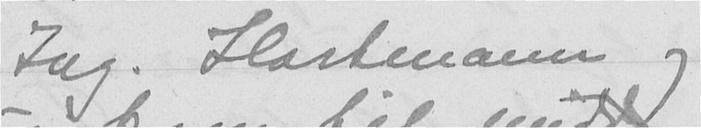Ing. Hartmann og
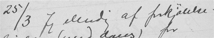25/3 Jeg elendig af forkjølelse.
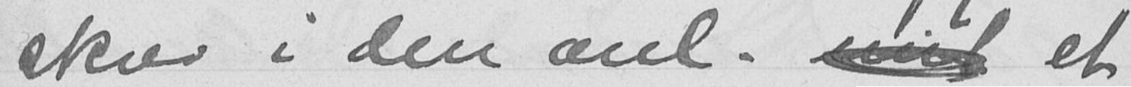skrev i den anl. mit et
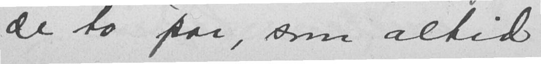de to par, som altid
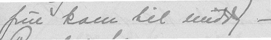frue kom til middag -
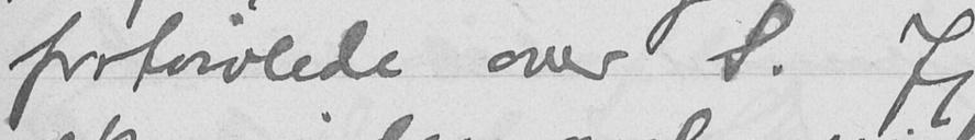fortvivlede over S. Jeg
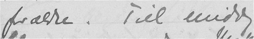forældre. Til middag
